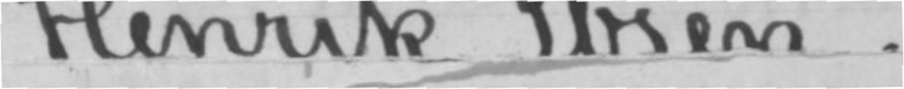Henrik Ibsen.
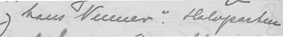og hans Venner". Halvparten
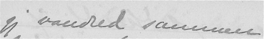jeg vandred sammen
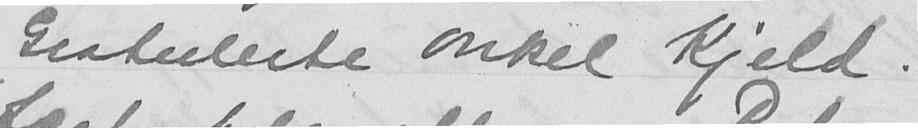Gratulerte onkel Kjeld.
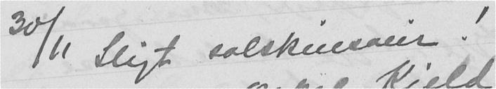30/11 Sligt solskinsveir!
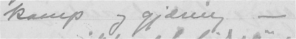kamp og gjærrig -
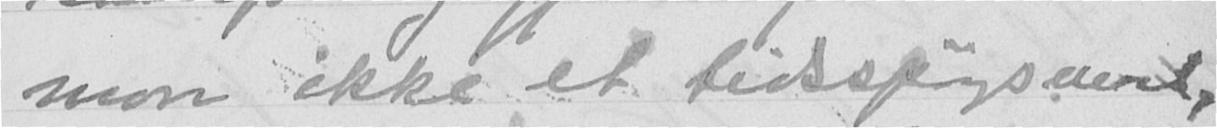mon ikke et tidsspørgsmaal,
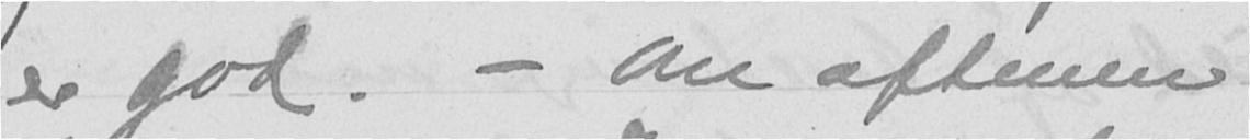er god. - Om aftenen
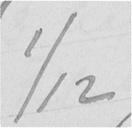1/12
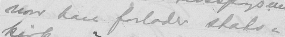naar han forlader stats-
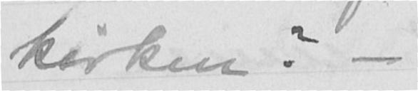kirken? -
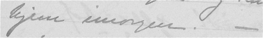hjem imorgen. -
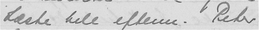Læste hele efterm. Peter
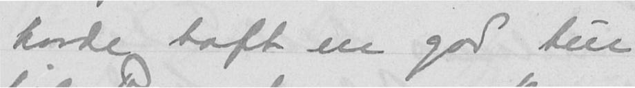havde haft en god tur
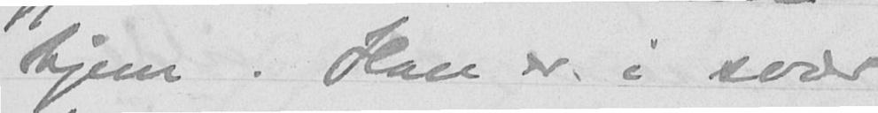hjem. Han er i svær
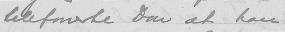telefonerte Don at han
